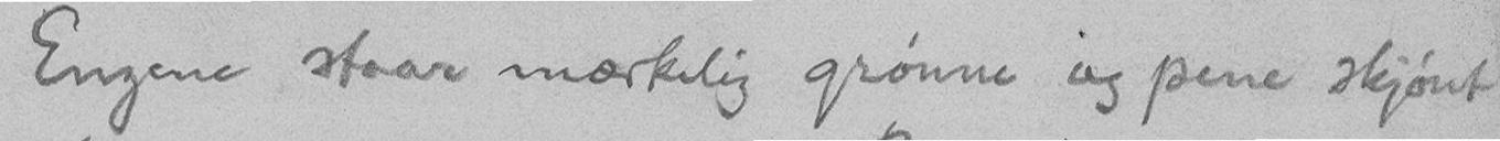Engene staar mærkelig grønne og pene skjønt
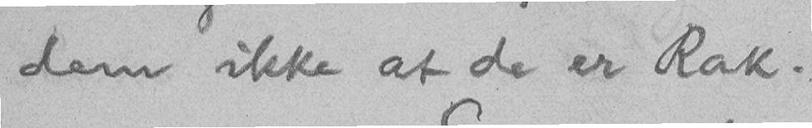dem ikke at de er Rak.
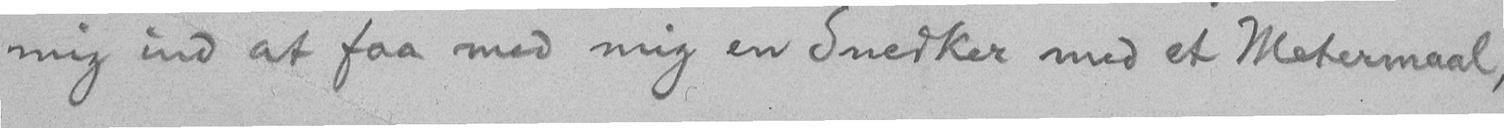mig ind at faa med mig en Snedker med et Metermaal,
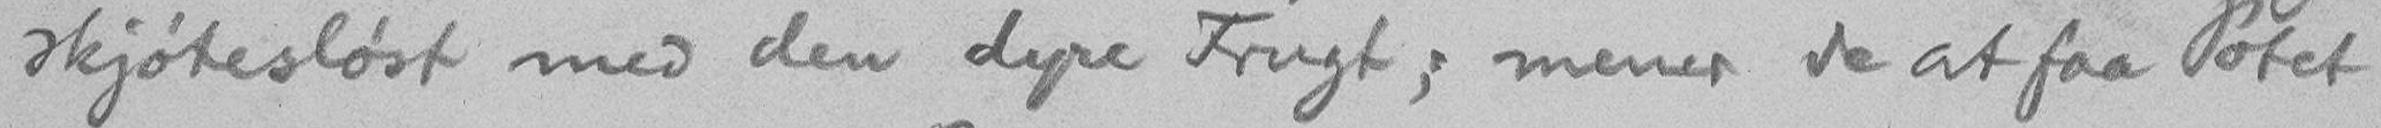skjøtesløst med den dype Frugt; mener de at faa Potet
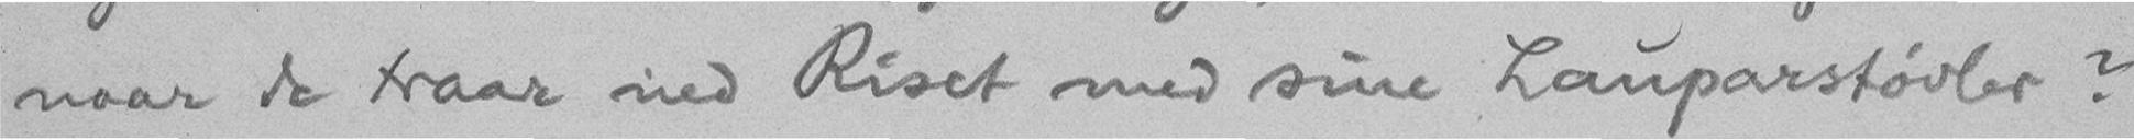naar de traar ned Riset med sine Lauparstøvler?
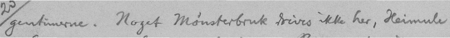gentimerne. Noget Mønsterbruk trives ikke her, Heimule
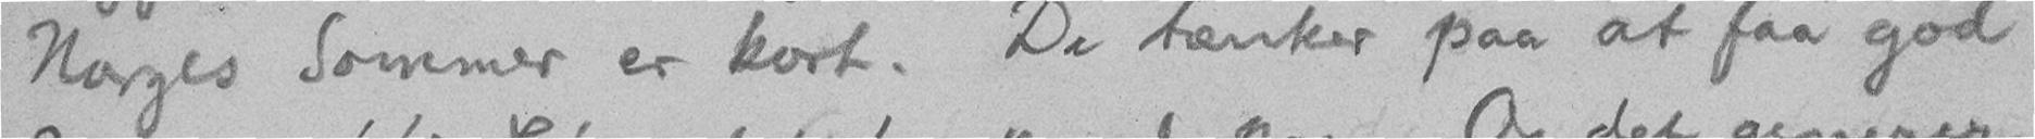Norges Sommer er kort. De tænker paa at faa god
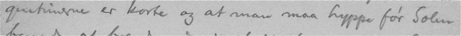gentimerne er korte og at man maa hyppe før Solen
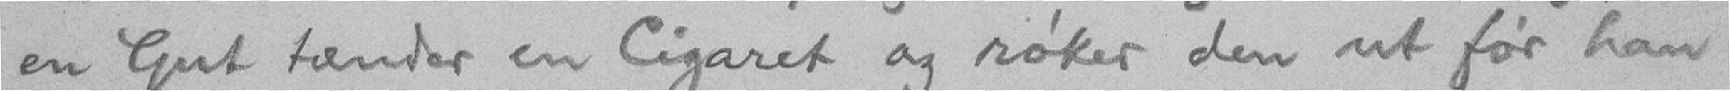en Gut tænder en Cigaret og røker den ut før han
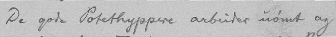De gode Potethyppere arbeider uømt og
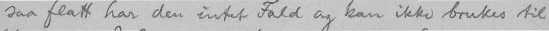saa flatt har den intet Fald og kan ikke brukes til
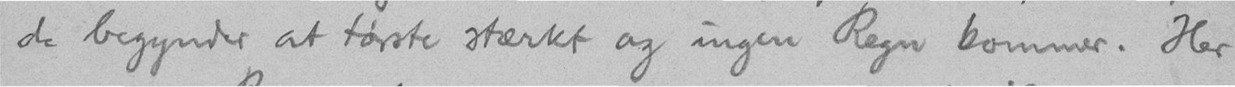de begynder at tørste stærkt og ingen Regn kommer. Her
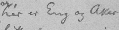her er Eng og Aker
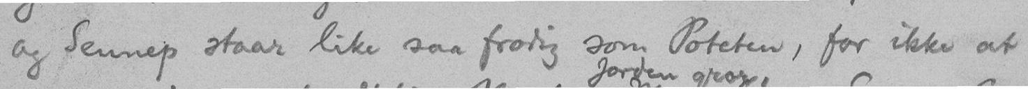og Sennep staar like saa frodig som Poteten, for ikke at
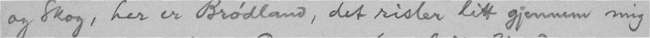og Skog, her er Brødland, det risler litt gjennem mig
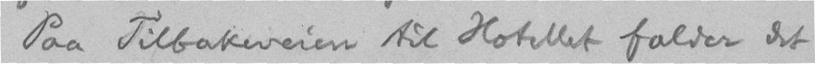Paa Tilbakeveien til Hotellet falder det
